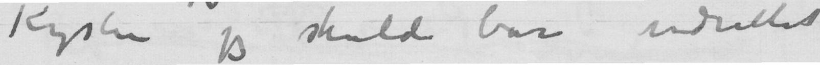Kysten jeg skulde bare udrettet
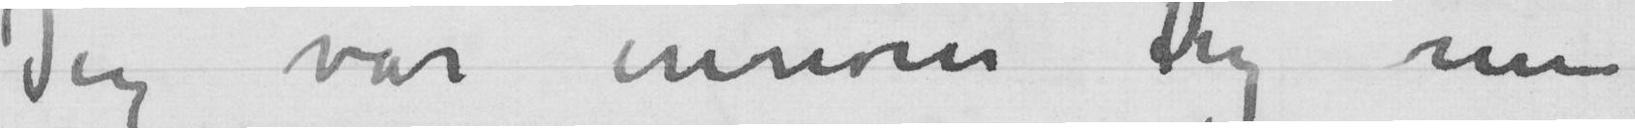Jeg var innom Dig men
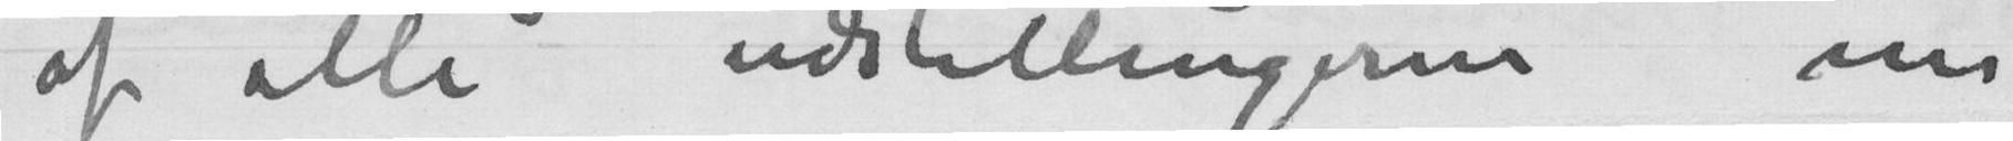af alle udstillingerne nu
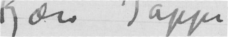Kjære Jappe
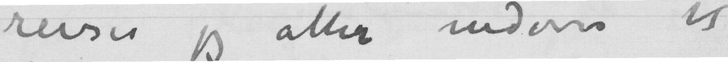reiser jeg atter nedover til
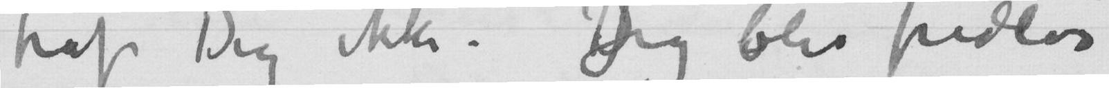traf Dig ikke. Jeg blir fredløs
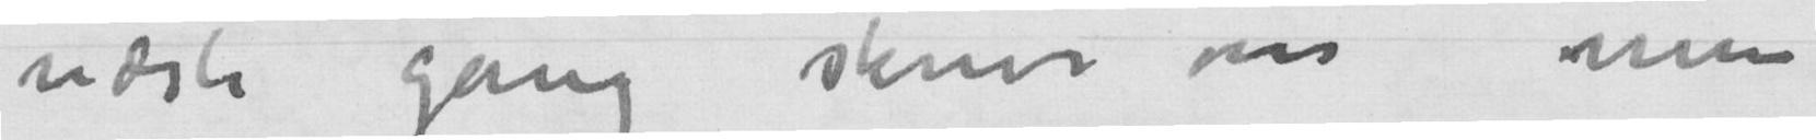næste gang skrive om min
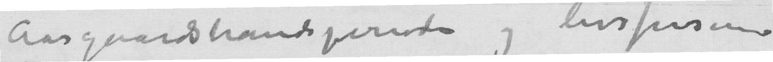Aasgaardstrandperiode og livsfrisen
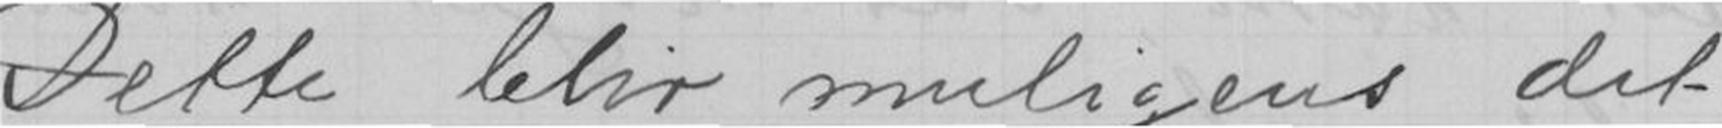Dette blir muligens det
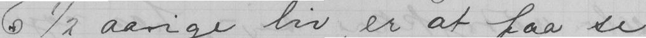6 1/2 aarige liv, er at faa se
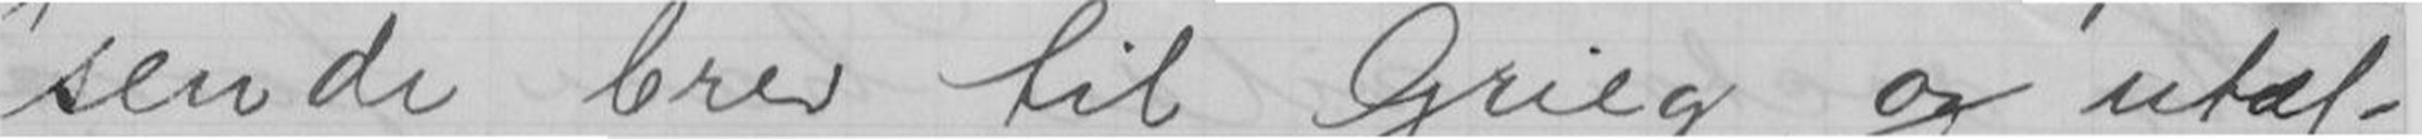sende brev til Grieg, og utal-
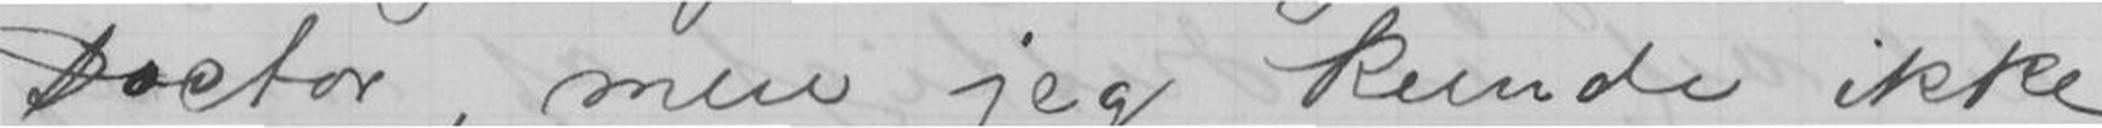Doctor, men jeg kunde ikke
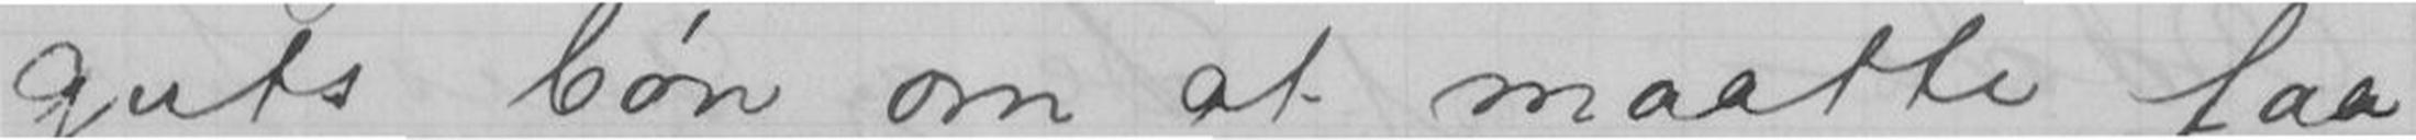guts bøn om at maatte faa
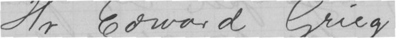Hr.Edwart Grieg!
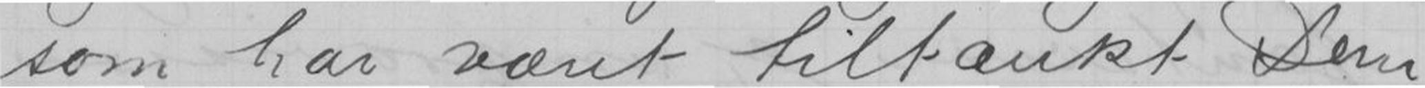som har været tiltænkt Dem
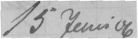15 Juni 06
